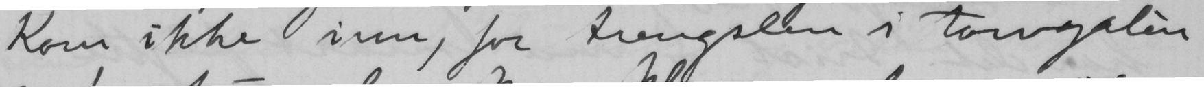Kom ikke inn, for trengslen i Torvgaten
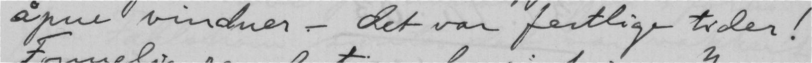åpne vinduer - det var festlige tider!
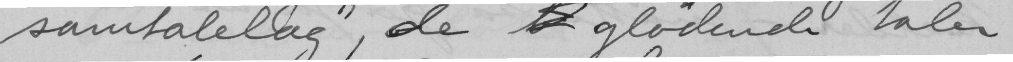samtalelag", de  glødende taler
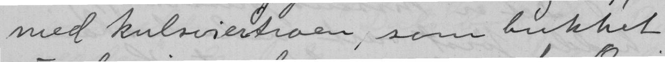med kulsviertroen, som bukket
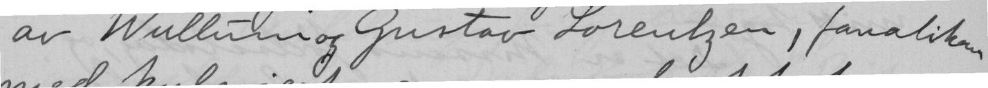av Wullum og Gustav Lorentzen, fanatikeren
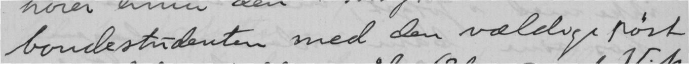bondestudenten med den vældige røst
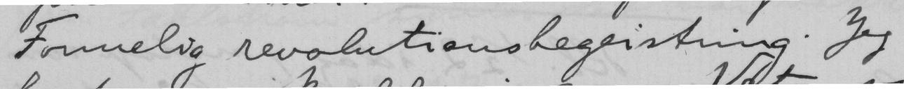Formelig revolutionsbegeistring. Jeg
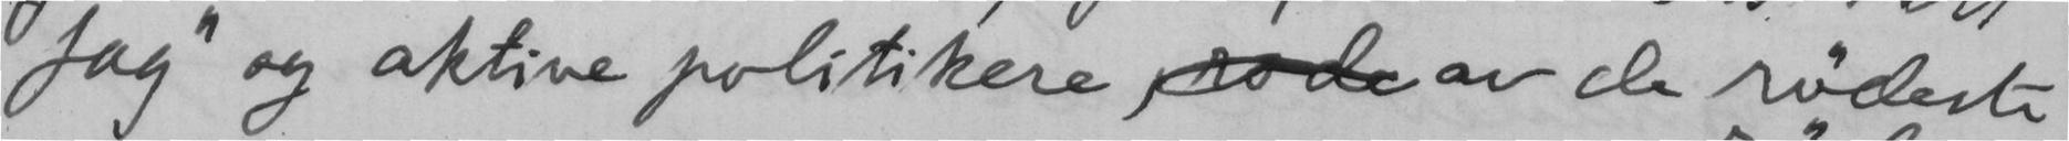fag" og aktive politikere røde av de rødeste
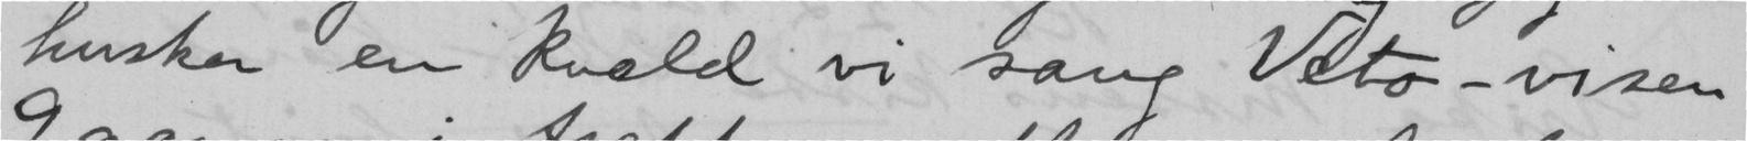husker en kveld vi sang Veto-visen
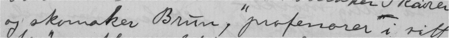og skomaker Brun, "professorer i sitt
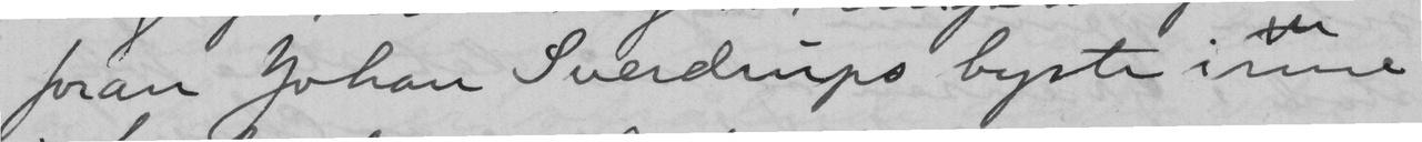foran Johan Sverdrups byste inne
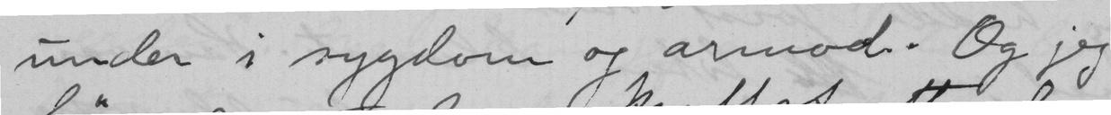under i sygdom og avmod. Og jeg
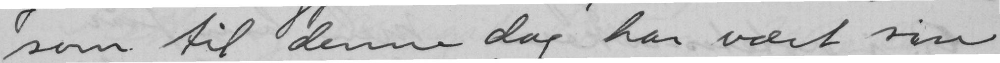som til denne dag har vært sin
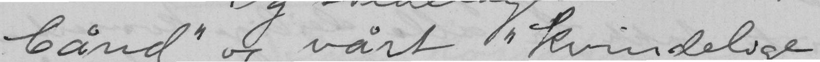bånd" og vårt "kvindelige
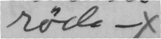røde - x
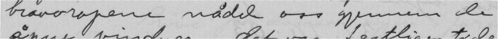bravoropene nåde oss gjennem de
